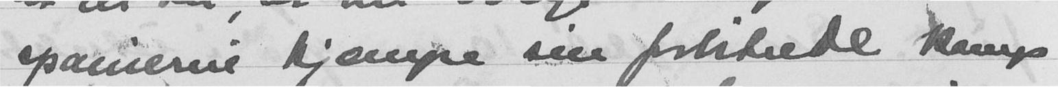spanierne kjempe sin forbitrede kamp
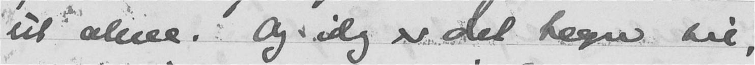ut alene. Og idag er det tegn til,
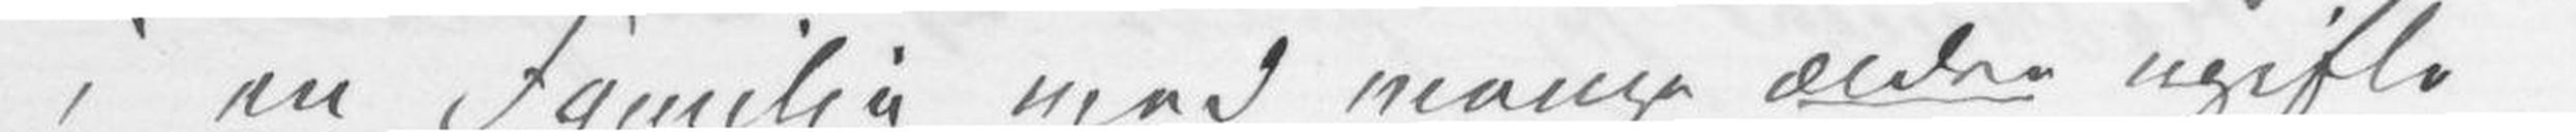i en Familie med mange ældre ugifte
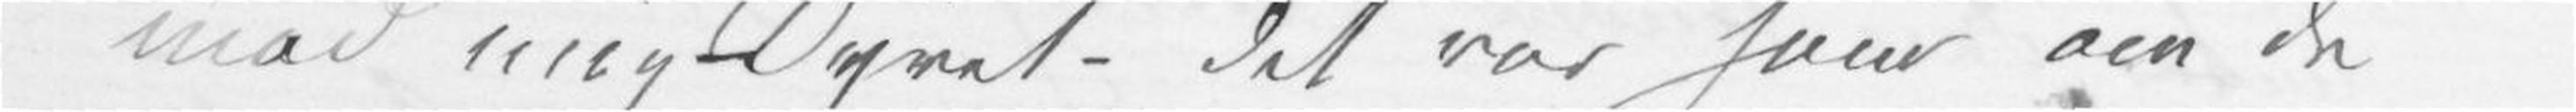mod mig Dyret. Det var som om de
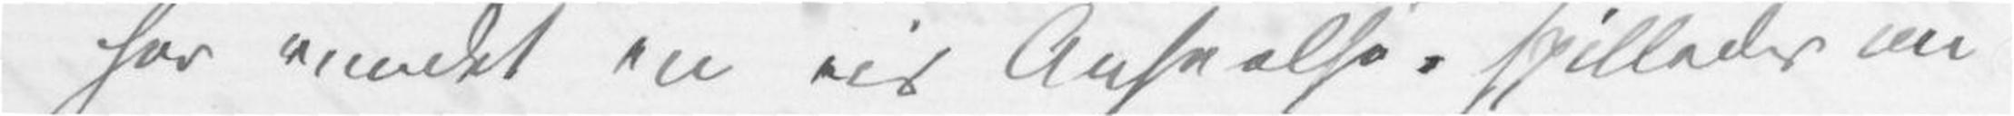har vundet en vis Anseelse – spilledes nu
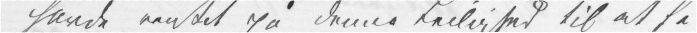havde ventet på denne Leilighed til at få
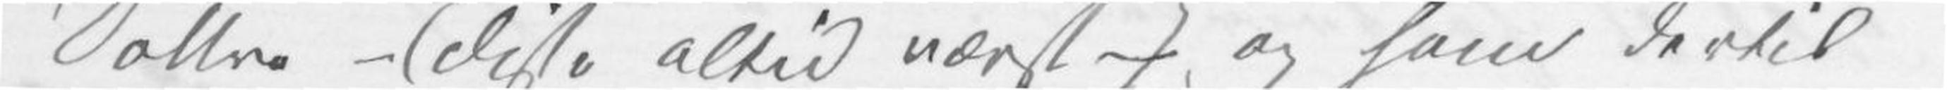Døttre – (disse altid værst) – og som dertil
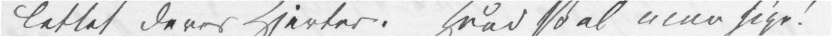lettet deres Hjerter. Hvad skal man sige!
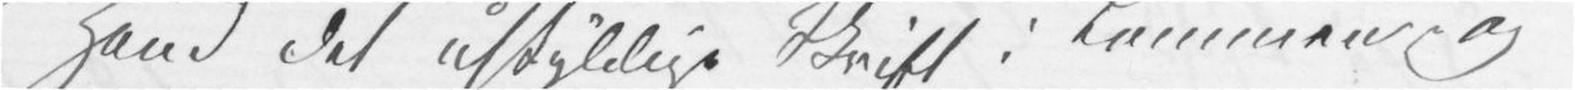Hånd det uskyldige Skrift i Lommen og
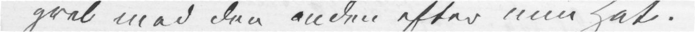greb med den andre efter min Hat.
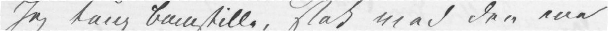Jeg taug bomstille, stak med den ene
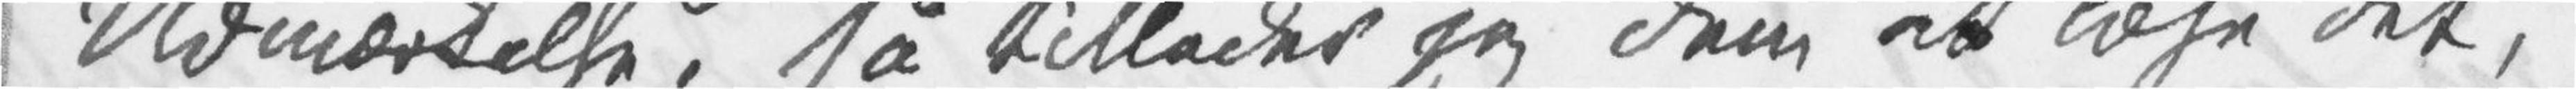Udmærkelse, så tillader jeg dem at læse det,
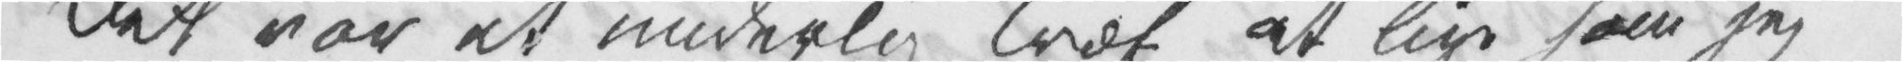Det var et underlig Træf at lige som jeg
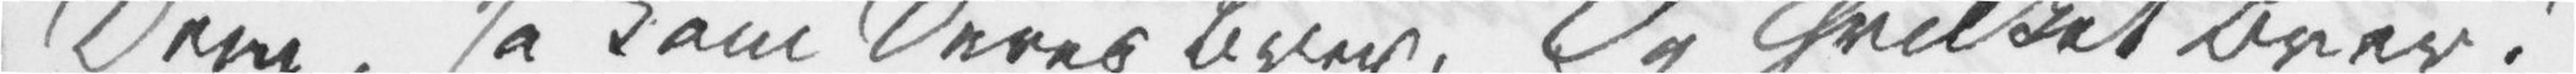Dem, så kom Deres Brev. Og hvilket Brev!
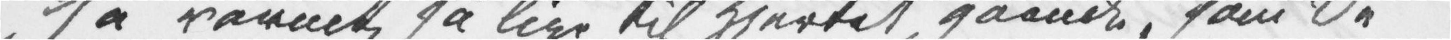Så varmt så lige til Hjertet gående, som De
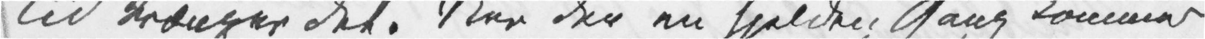Tid trænger det. Når der en sjelden Gang kommer
